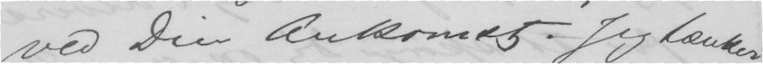ved Din Ankomst. Jeg tænker
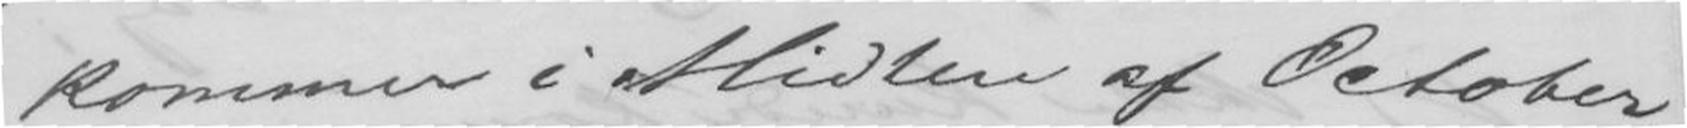kommer i Midten af October,
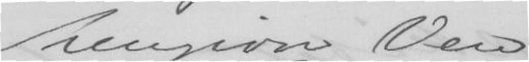hengivne Ven
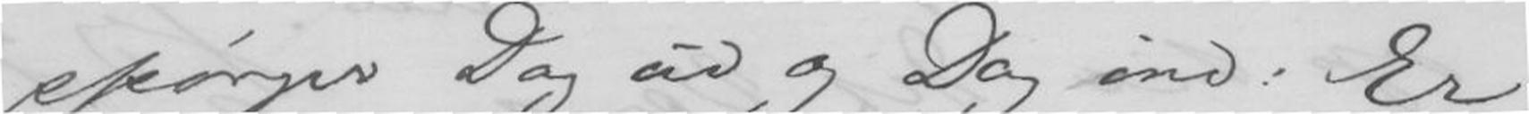spørger Dag ud og Dag ind: Er
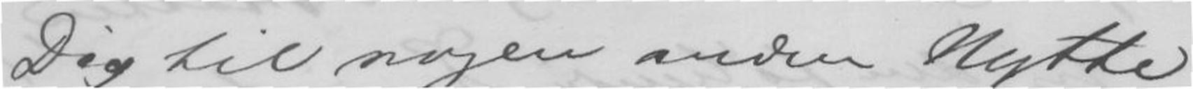Dig til nogen anden Nytte
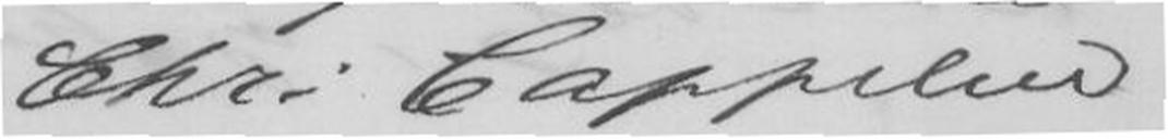Chr. Cappelen
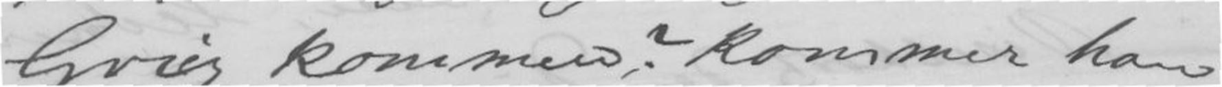Grieg kommen? kommer han
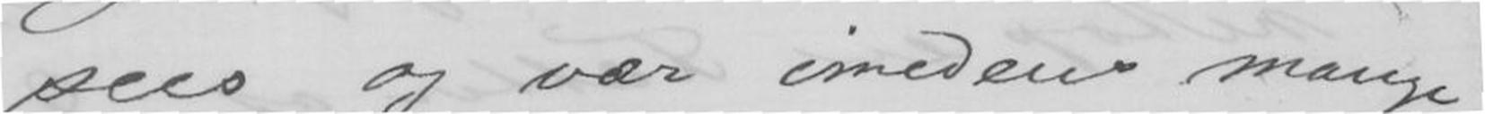sees og vær imedens mange
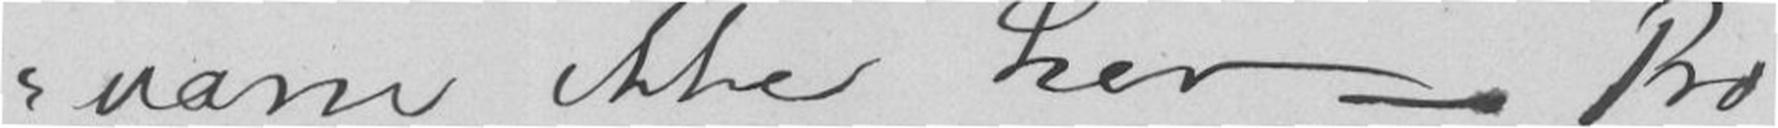værre ikke her. Pro
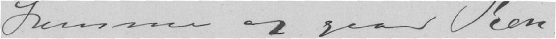komme og give Kon
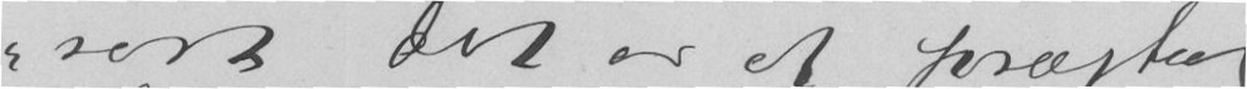sert det er et prægtig
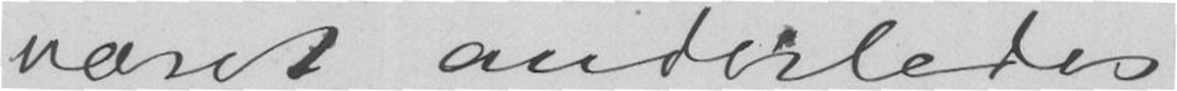været enderledes
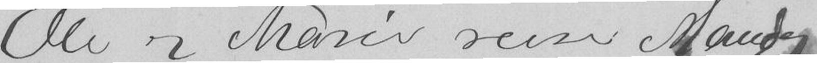Ole og Marie reiser Mandag
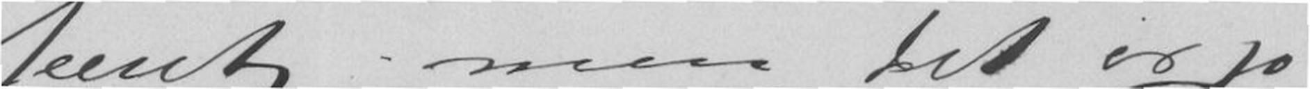seent men det er jo
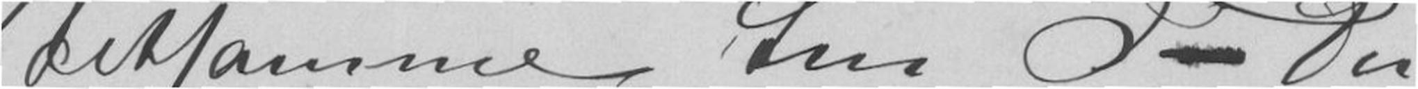detsamme tror I - Du
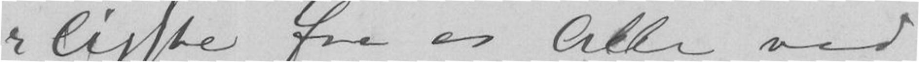ligste fra os Alle ved
